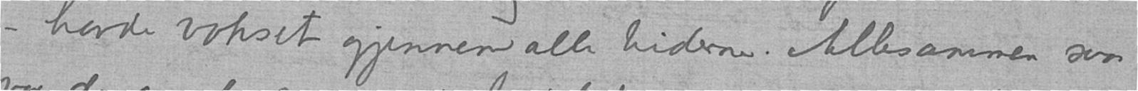- havde vokset gjennem alle tiderne. Allesammen saa
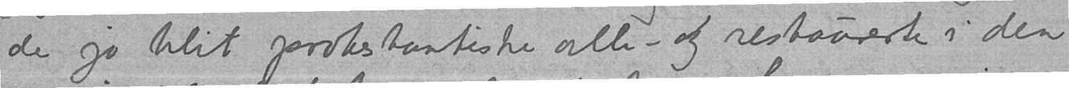de jo blit protestantiske alle - og restaurerte i den
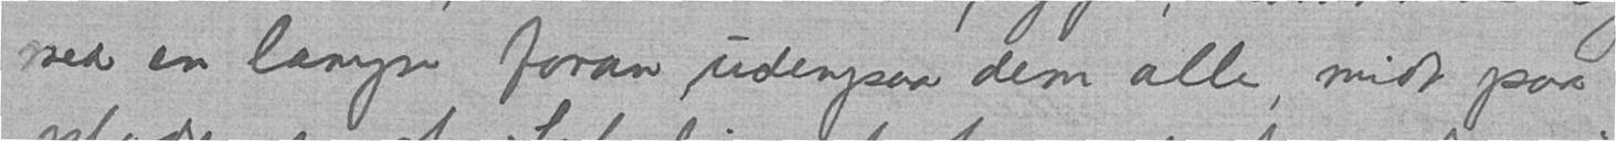med en lampe foran udenpaa dem alle, midt paa
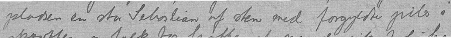pladsen en stor Sebastian af sten med forgyldte piler i
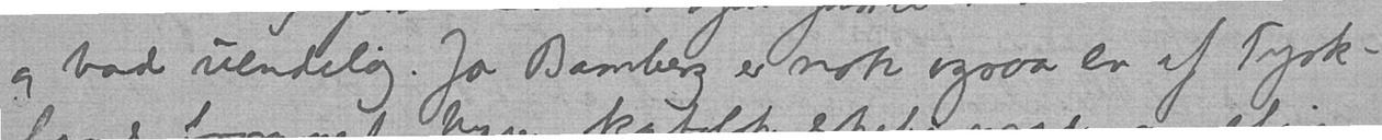og bad uendelig. Ja Bamberg er nok ogsaa en af Tysk-
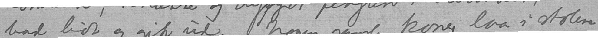bad lidt og gik ud. Nogen gamle koner laa i stolene
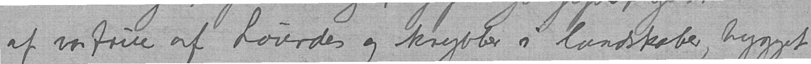af vorfrue af Lourdes og krybber i landskaber, bygget
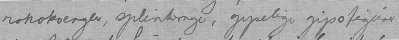rokokoengler, splinternye, gyselige gipsfigurer
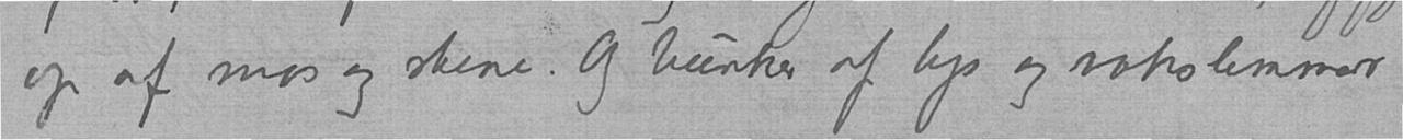op af mos og stene. Og bunker af lys og vokslemmer
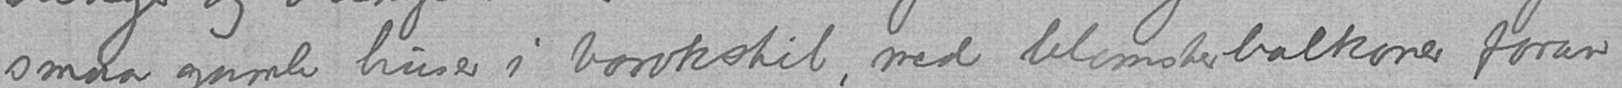smaa gamle huser i barokstil, med blomsterbalkoner foran
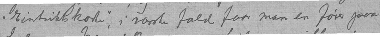"Eintrittskarte", i værste fald faar man en fører paa
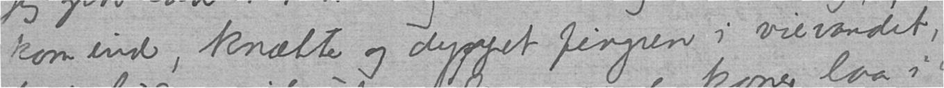kom ind, knælte og dyppet fingren i vievandet,
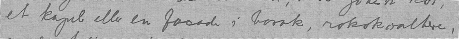et kapel eller en facade i barok, rokokoaltere,
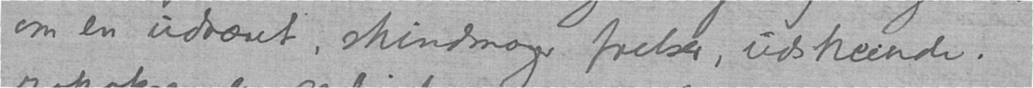om en udværet, skindmager frelser, udskeiende
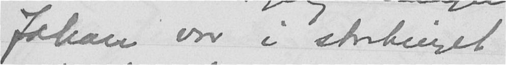Johan var i stortinget
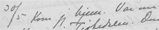30/5 Kom jeg hjem. Var om
